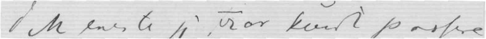dette eneste jeg tror kunde passer
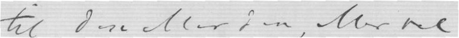til den eller den, eller vel
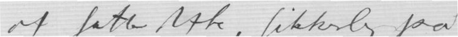at sætte dette, sikkerlig på
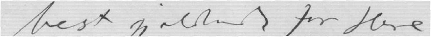best gjældende for flere
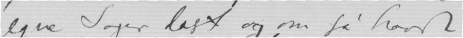egne Sager læst og, om så havde
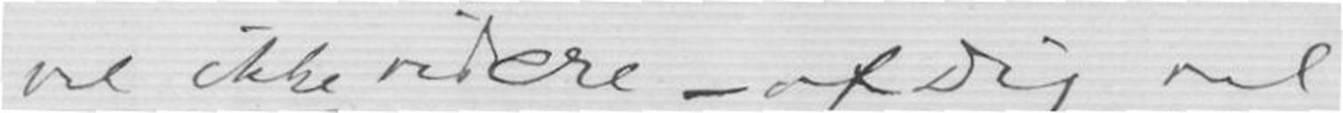vil ikke videre - af Dig vil
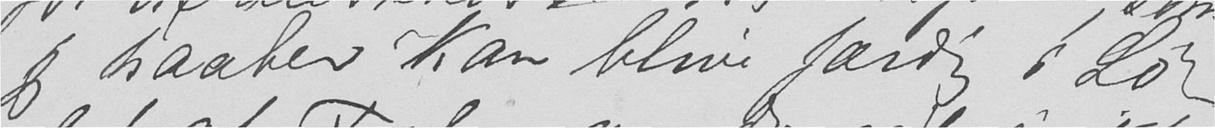jeg haaber kan blive færdig i Lø-
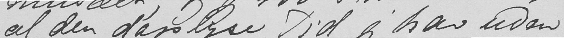al den dagslyse Tid jeg har uden
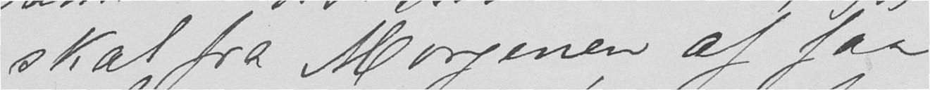skal fra Morgenen af faa
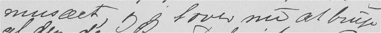musæet, og jeg lover nu at bruge
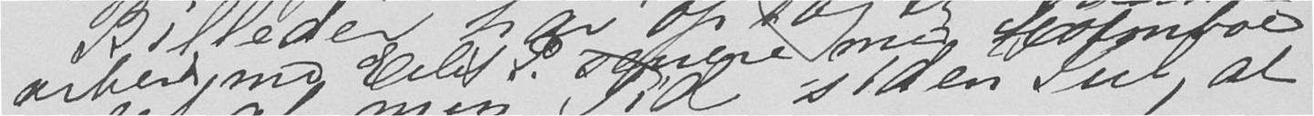arbeid med Eilif P. senere med Holmboe
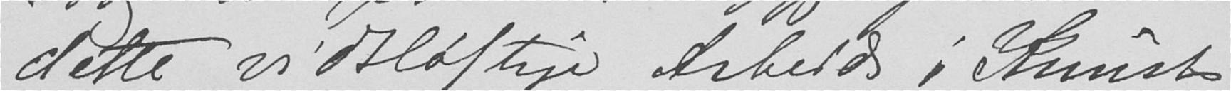dette vidtløftige Arbeide i Kunst-
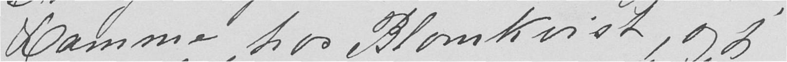Ramme hos Blomkvist, og jeg
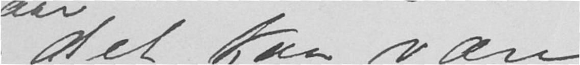det kan være
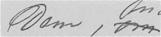Dem, om
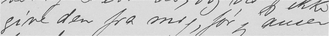give den fra mig, før jeg anser
